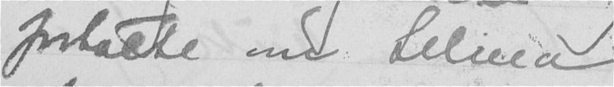fortalte om Selma
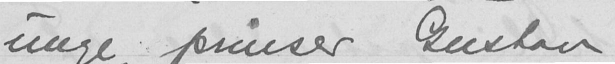unge prinser Gustav
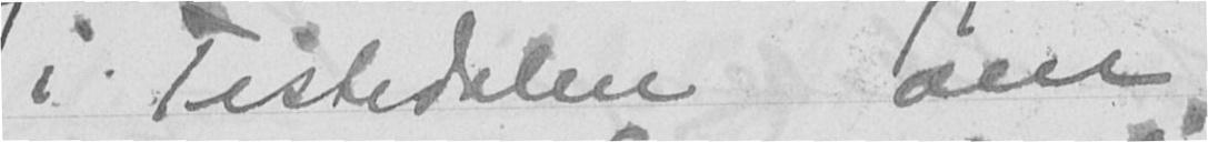i Tistedalen om
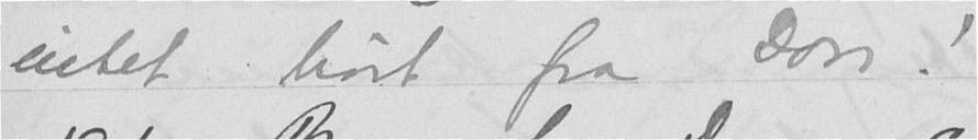intet hørt fra Don!
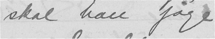skal han jage
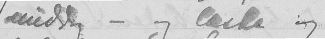middag - og læste og
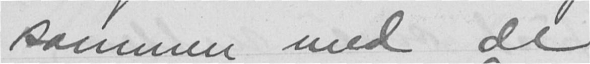sammen med de
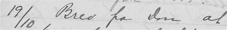19/10 Brev fra Don at
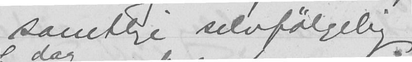samtlige selvfølgelig.
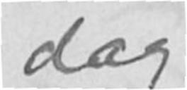dag
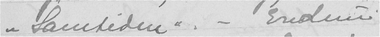"Samtiden". - Endnu
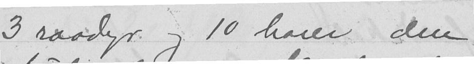3 raadyr og 10 harer den
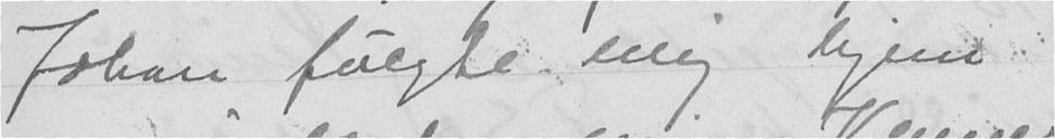Johan fulgte mig hjem
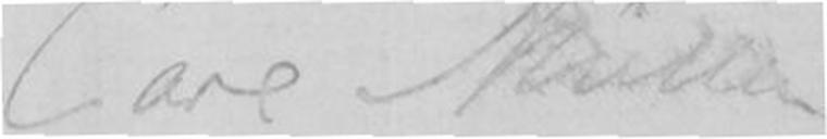Care Müller
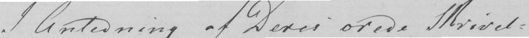I Anledning af Deres ærede Skrivel-
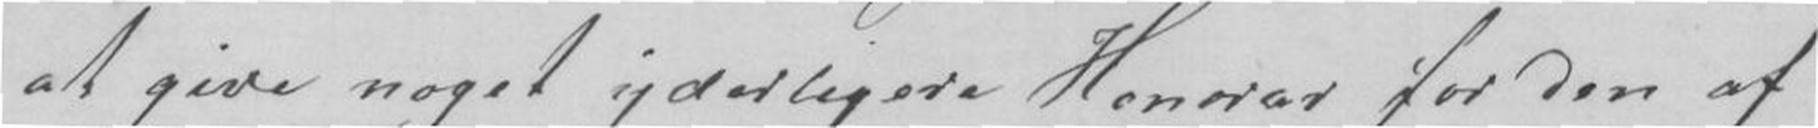at give noget yderligere Honorar for den af
